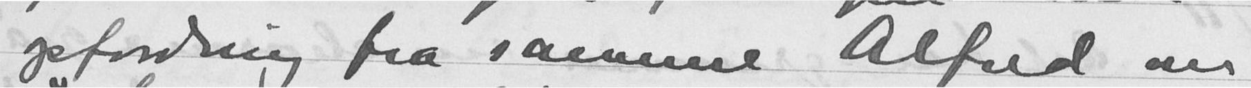opfordring fra samme Alfred om
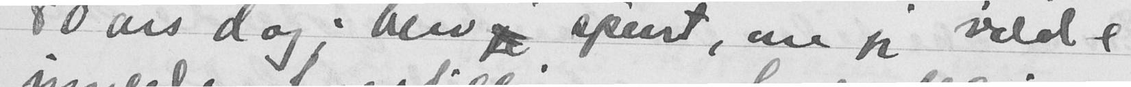80 års dag  blev spurt, om jeg vilde
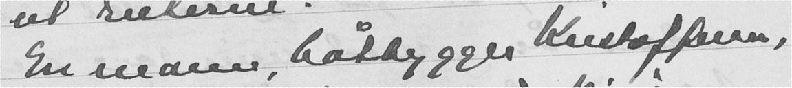En mann, båtbygger Kristoffersen,
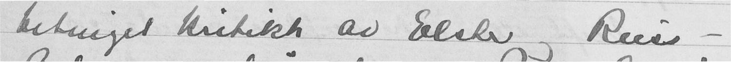betinget kritikk Av Elster og Reiss -
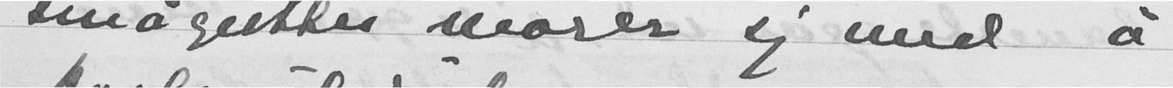smågutter morer sig med å
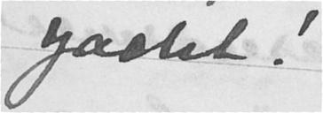yacht!
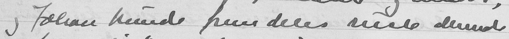og Johan kunde fremdeles reise derinde
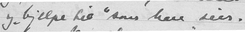og "hjelpe til" som han sier.
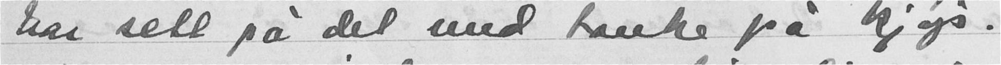har sett på det med tanke på kjøp.
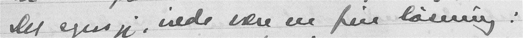Det syns jeg, vilde være en fin løsning:
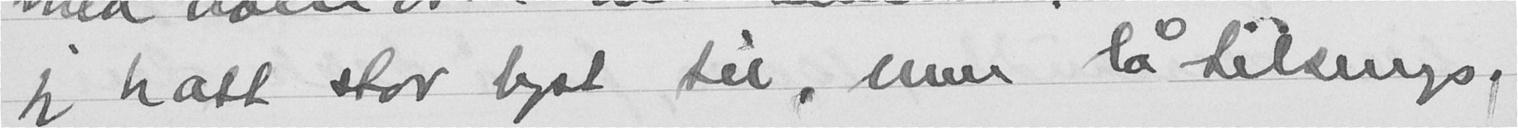jeg hatt stor lyst til, men lå tilsengs.
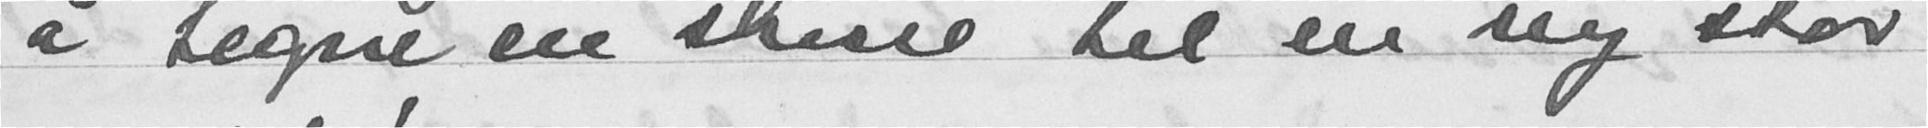å tegne en skisse til en ny stor
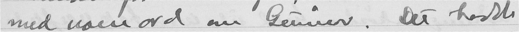med noen ord om Gunnar. Det hadde
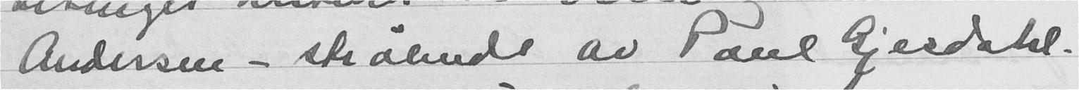Andersen - strålende av Paul Gjesdahl.
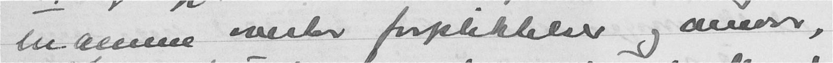mennene overtar forpliktelser og ansvar,
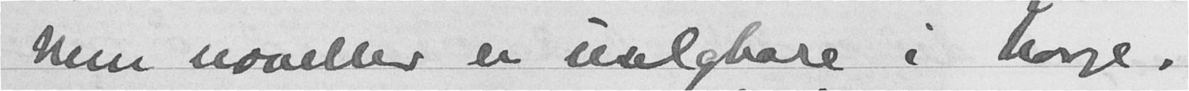Men noveller er uselgbare i Norge,
